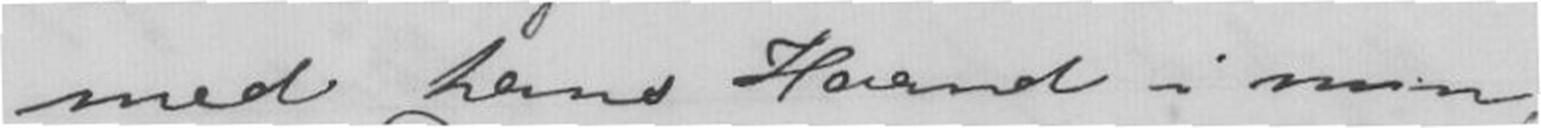med hans Haand i min,
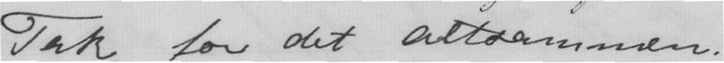Tak for det altsammen!
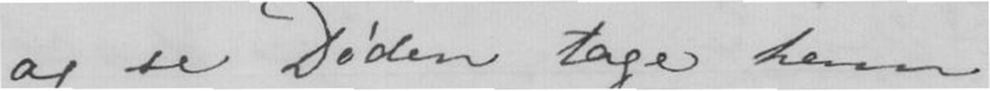og se Døden tage ham
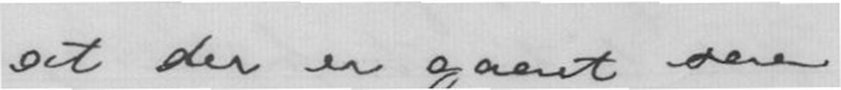at der er gaaet dere
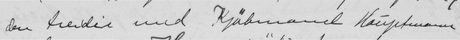den treidie med Kjøbmand Hauptann
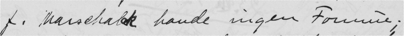f. Marschalk havde ingen Formue.
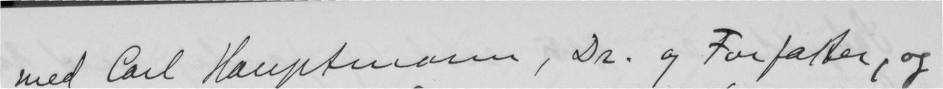med Carl Hauptmann, Dr. og Forfatter, og
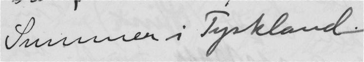Summer i Tyskland.
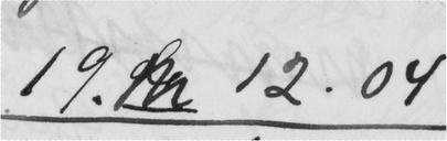19. 12.04
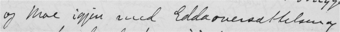og Moe igjen med Eddaoversættelsen og
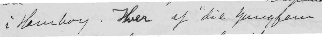i Hamburg. Hver af "die yungfere
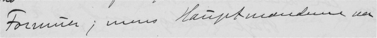Formuer; mens Hauptmandene var
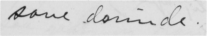sove derinde.
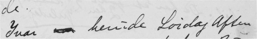Ivar  herude Lørdag Aften
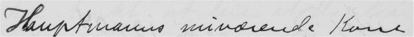Hauptmanns nuværende Kone
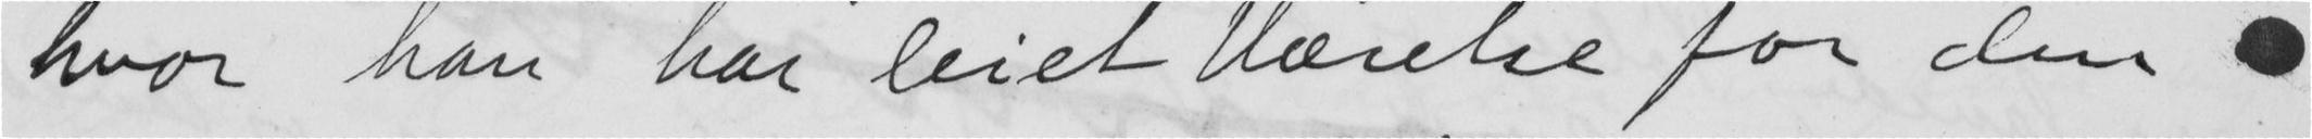hvor han har leiet Værelse for den
